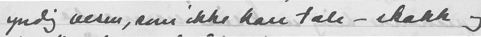yndig vesen, som ikke kan tale - skakk og
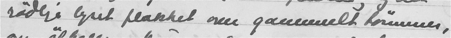rødlige lyset flakket som gammelt tømmer,
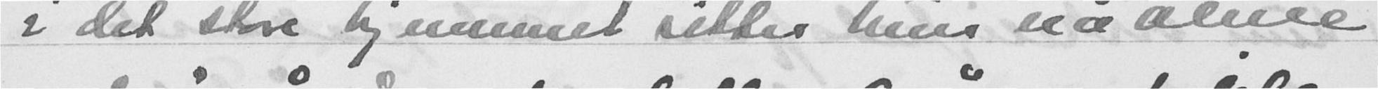i det store hjemmet sitter hun nå alene
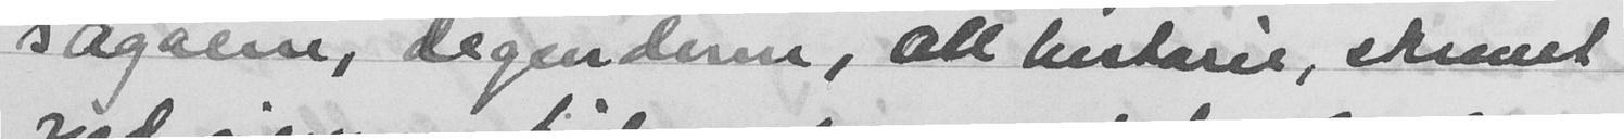sagaene, legendene, all historie, skrevet
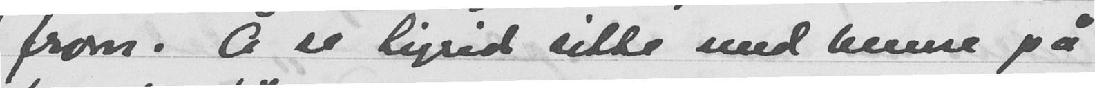from. Å se Sigrid sitte med henne på
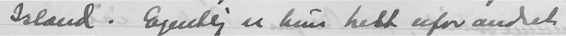Island. Egentlig er hun helt uforandret
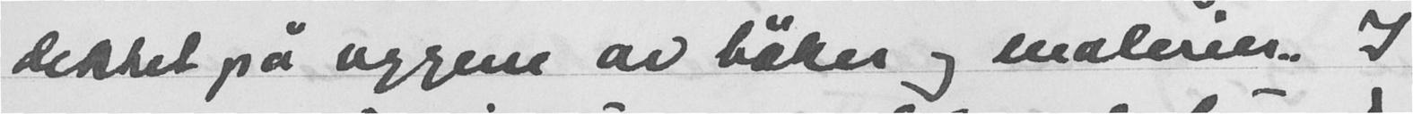dekket på veggene av bøker og malerier. I
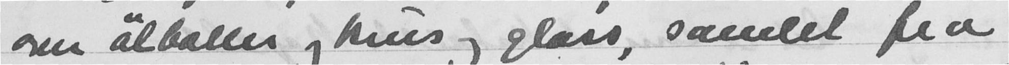over ølhallen og krus og glass, samlet fra
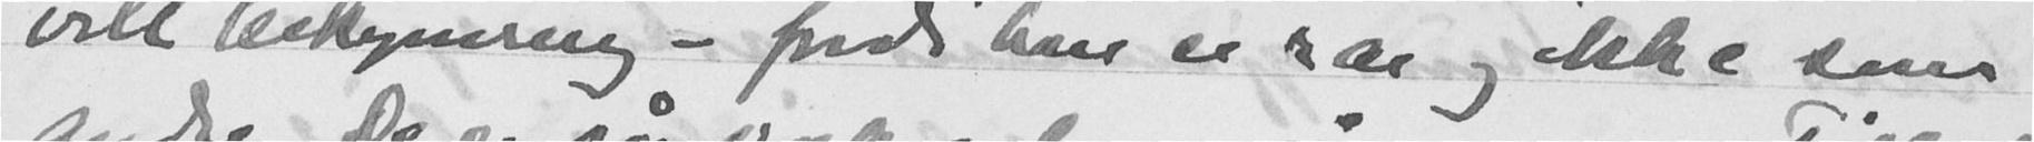vill bekymring - fordi han er rar og ikke som
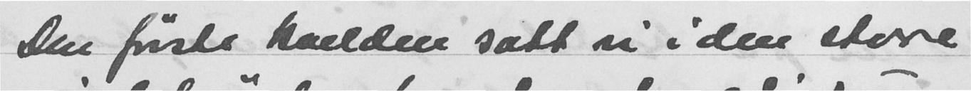Den første kvelden satt vi i den store
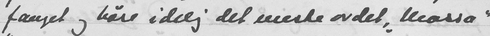fanget og høre idelig det eneste ordet, "Massa"
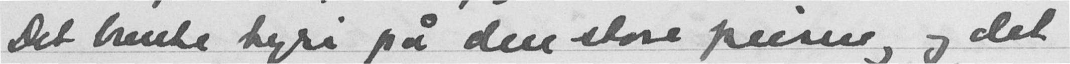Det brente tyru på den store peisen, og det
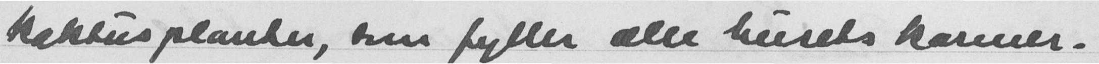kaktusplanter, som fyller alle husets karmer.
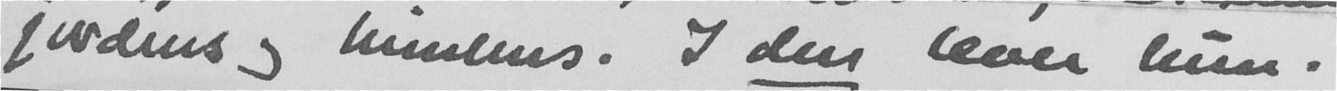jordens og himlens. I den lever hun.
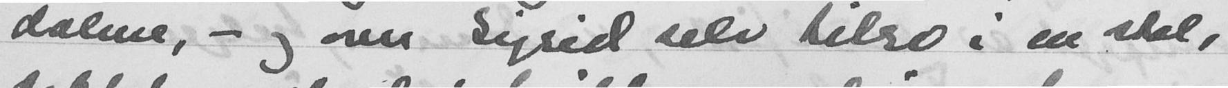dalene, - og over sigrid selv tilso i en skål,
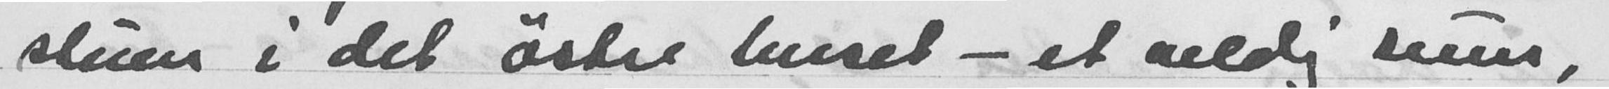stuen i det østre huset - et veldig hus,
